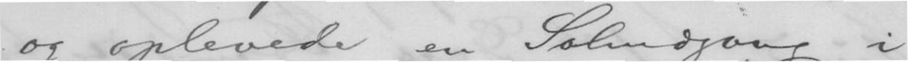og oplevede en Solnedgang i
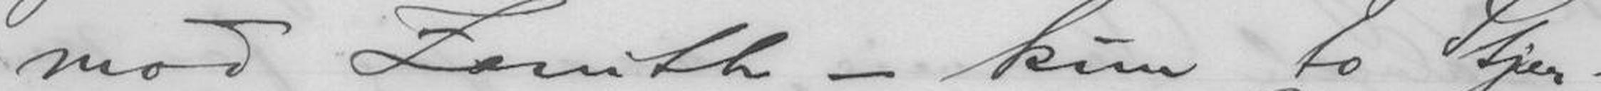mod Zenith - kun to Stjer-
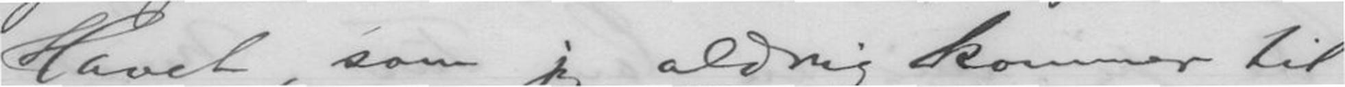Havet, som jeg aldrig kommer til
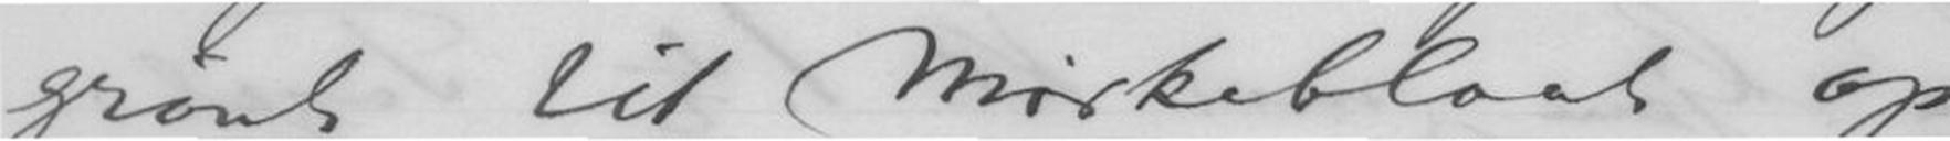grønt til Mørkeblaat op
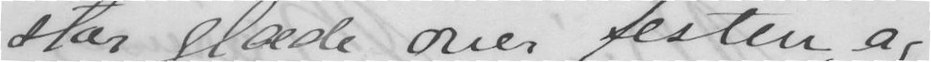stor glæde over festen, og
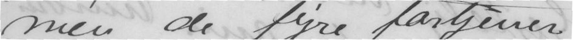men de fyre fortjener
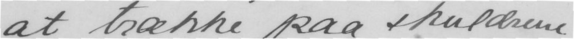at trække paa skuldrene
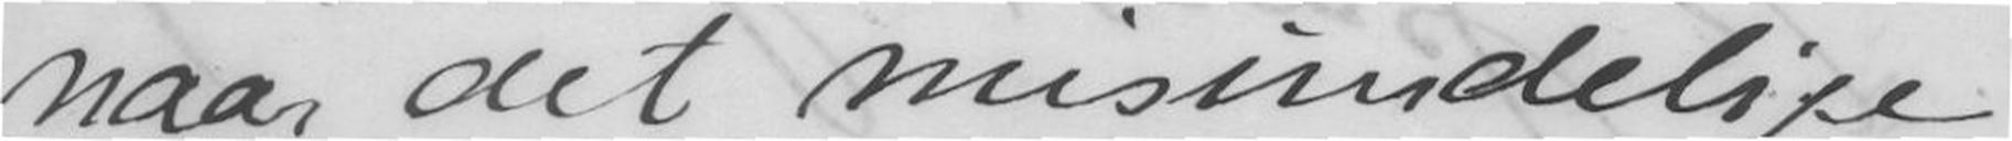naar det misundelige
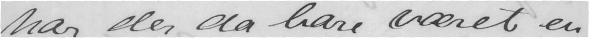har der da bare været en
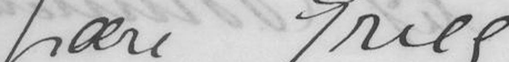Kjære Grieg
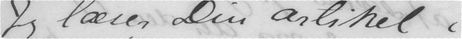Jeg læser Din artikel i
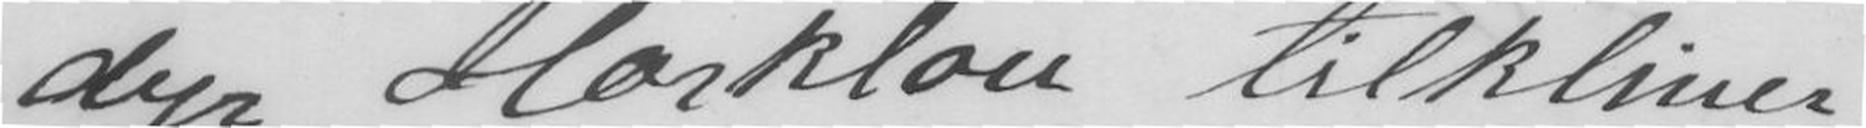dyr Horklou tilkliner
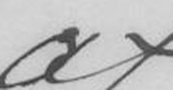af.
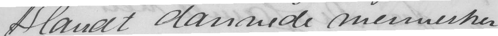Blandt dannede mennesker
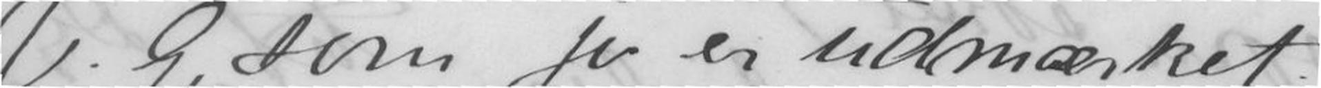V. G. som jo er udmærket,
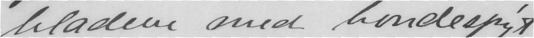bladene med bondespyt,
